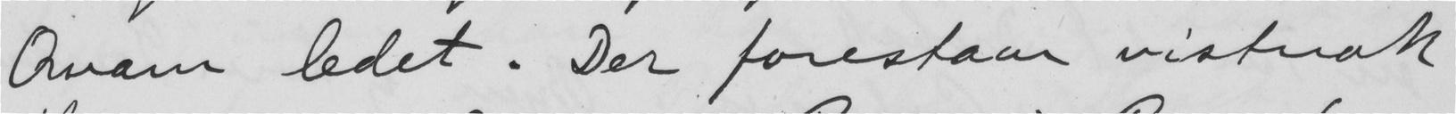Qvam ledet. Der forestaar vistnok
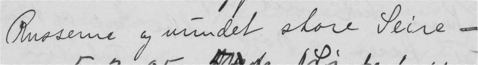Russerne og vundet store Seire -
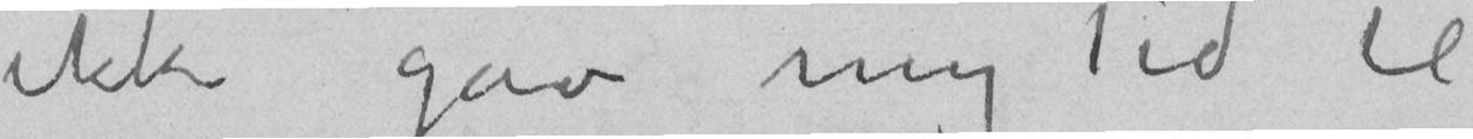ikke gav mig Tid til
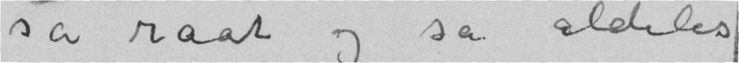så raat og så aldeles
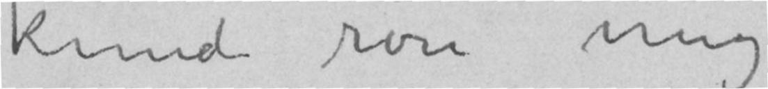kunde røre mig
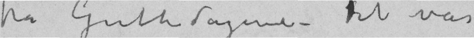fra Guttedagerne – Det var
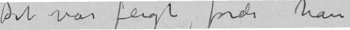Det var feigt, fordi han
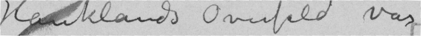Hauklands Overfald var
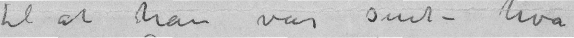til at han var sint – hva
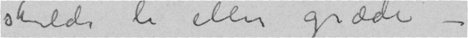skulde le eller græde –
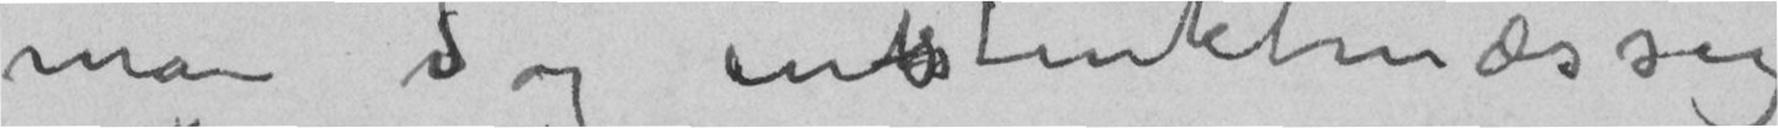man dog instinktmæssig
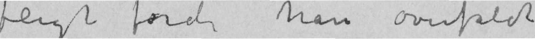feigt fordi han overfaldt
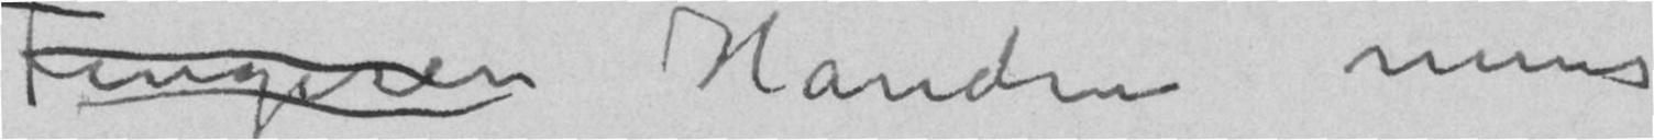Fingeren Hånden mens
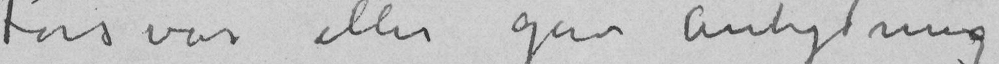Forsvar eller gav Antydning
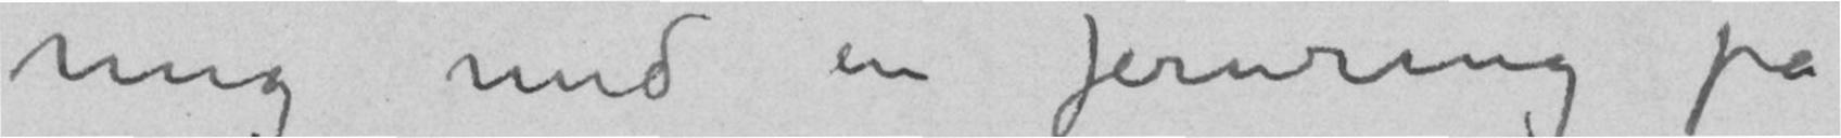mig med en jernring på
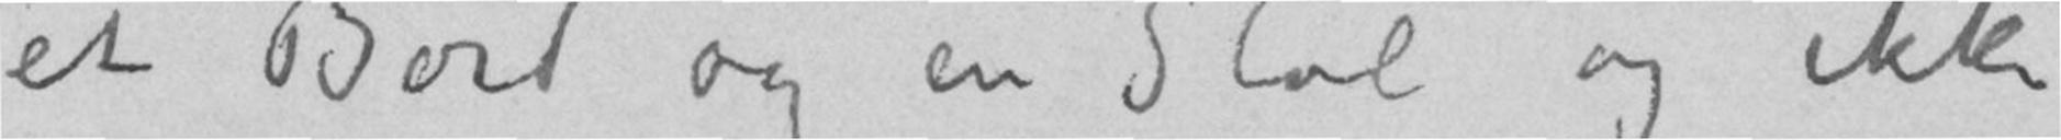et Bord og en Stol og ikke
